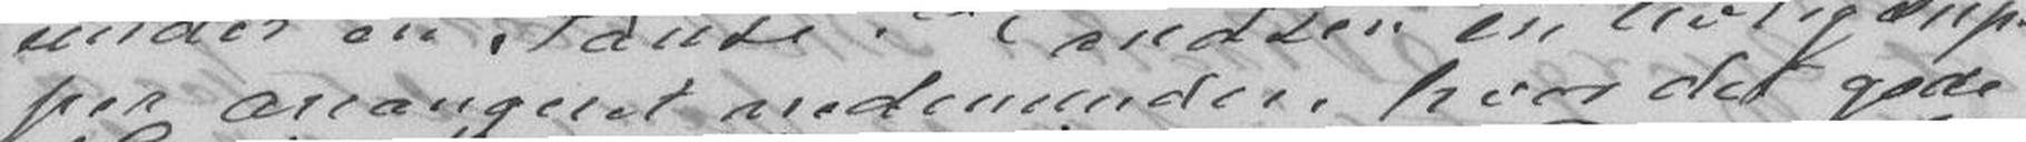per arrangeret nedenunder, hvor det gode
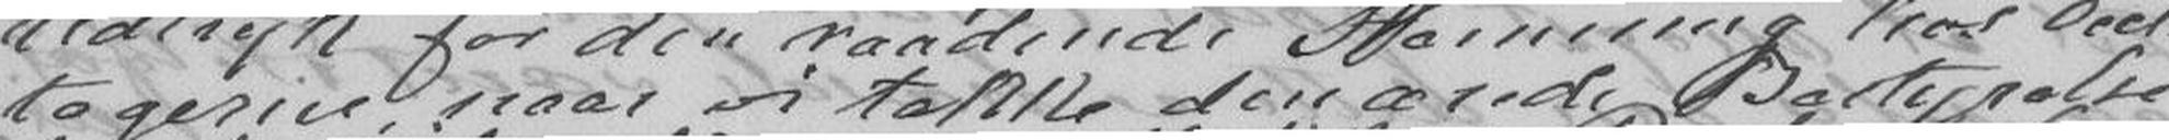tagerne naar vi takke den ærede Bestyrelse
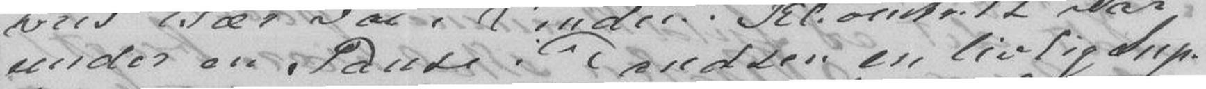under en Pause i Dandsen en livlig Sup-
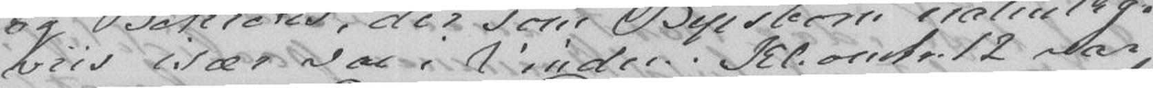viis især var i Vinden. Kl. omtr.12 var
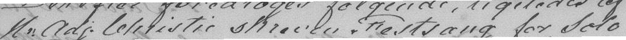Hr Adj. Christie skreven Festsang for Solo
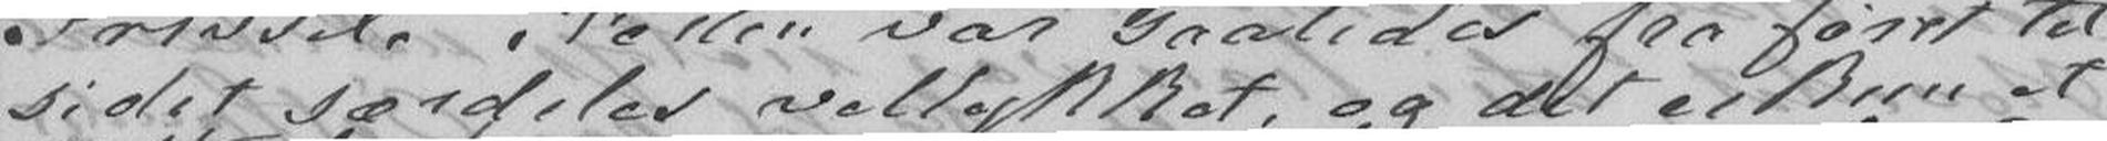sidst særdeles vellykket, og det er kun et
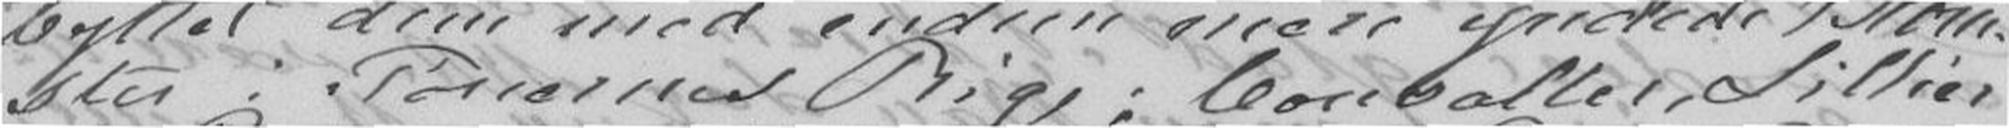ster i Tónernes Rige; Convaller, Lillier
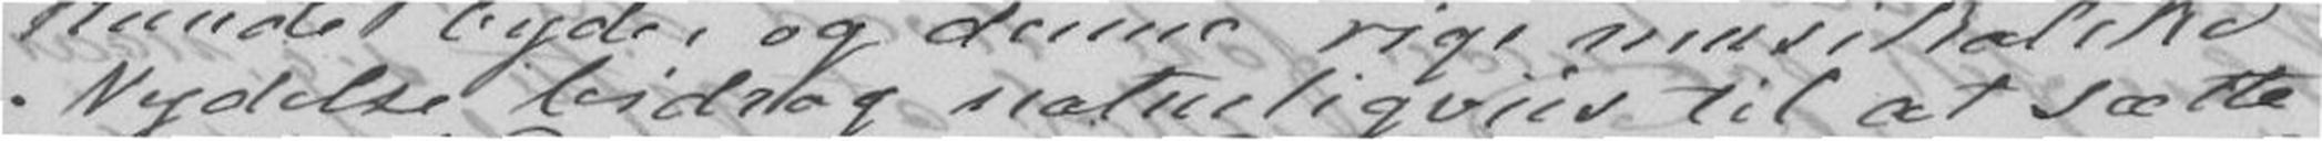Nydelse bidrog naturligviis til at sætte
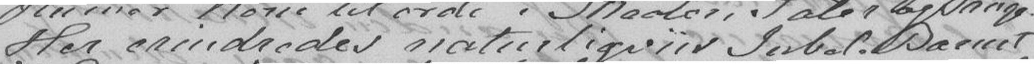Her erindredes naturligviis Jubel. Barnet
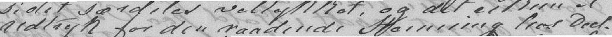Udtryk for den raadende Staæmning hos Deel-
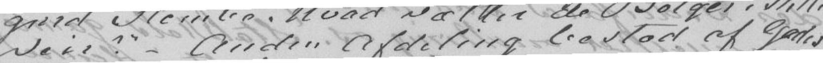veir?" Anden Afdeling bestod af Gades
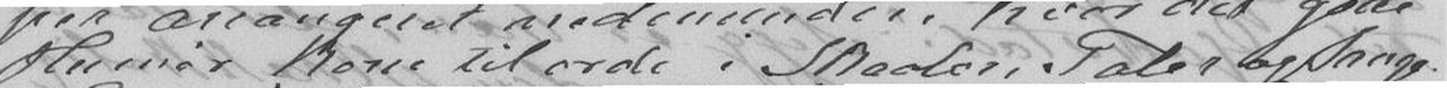Humør kom til orde i Skaaler, Taler og Sange.
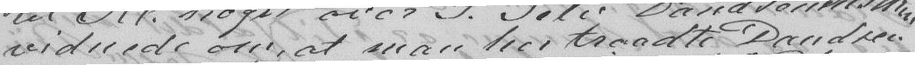vidnede om, at man her traadte Dandsen
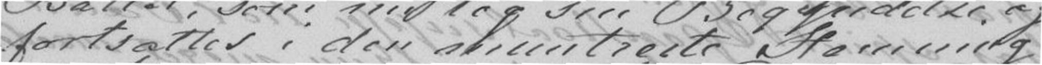fortsættes i den muntreste Stemning
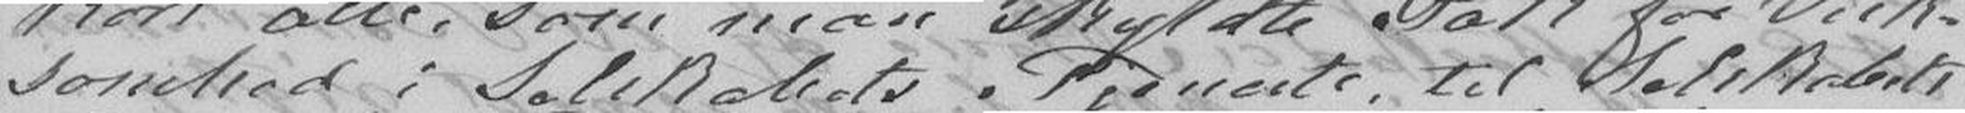somhed i Selskabets Tjeneste til Selskabets
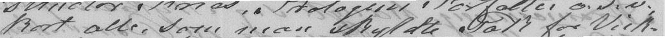kort alle, som man skyldte Tak for Virk-
